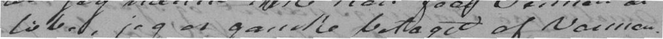løbe, jeg er ganske betaget af Varmen.
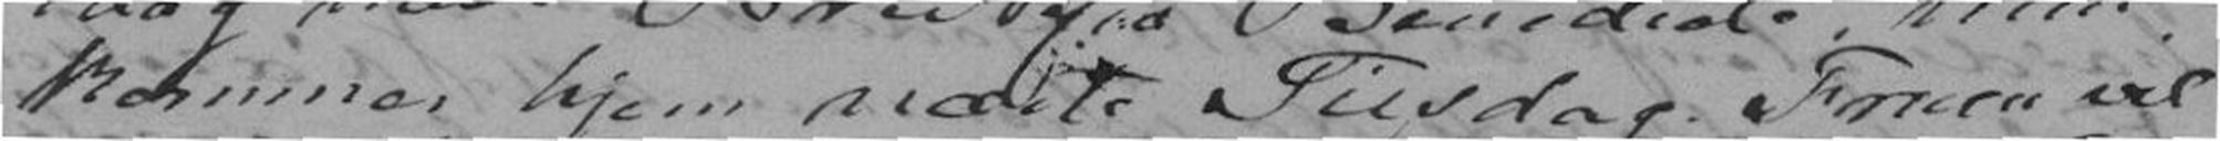kommer hjem næste Tirsdag. Fruen vil
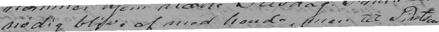nødig blive af med hende, men til Pintsen
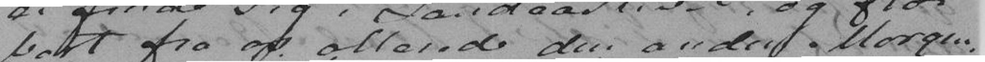bort fra os allerede den anden Morgen,
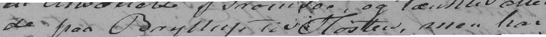de paa Bryllup til Høsten, men har
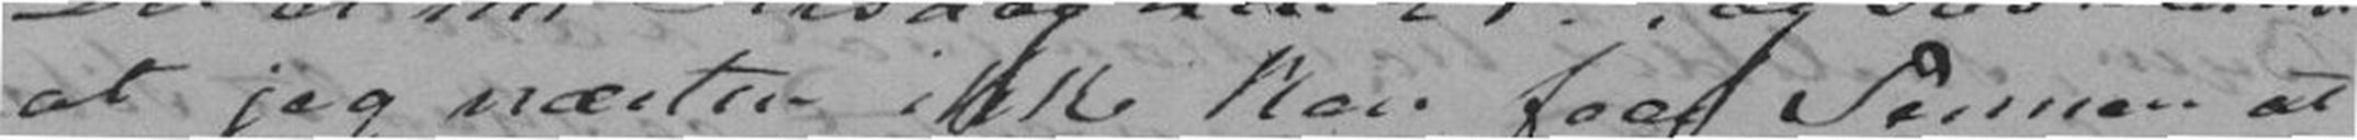at jeg næsten ikke kan faa Pennen at
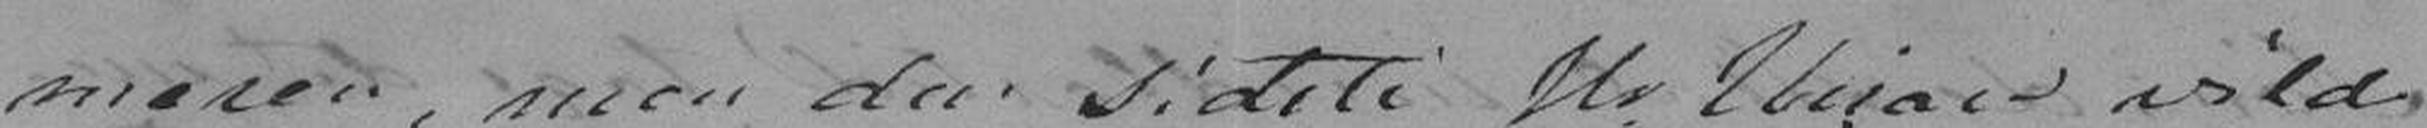meren, men den sidste Hr Urian vilde
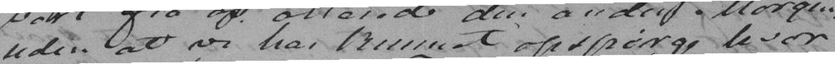uden at vi har kunnet opspørge hvor
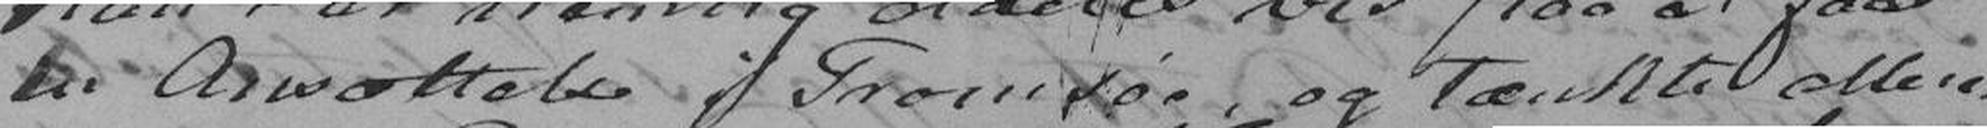en Ansættelse i Tromsøe, og tænkte allere-
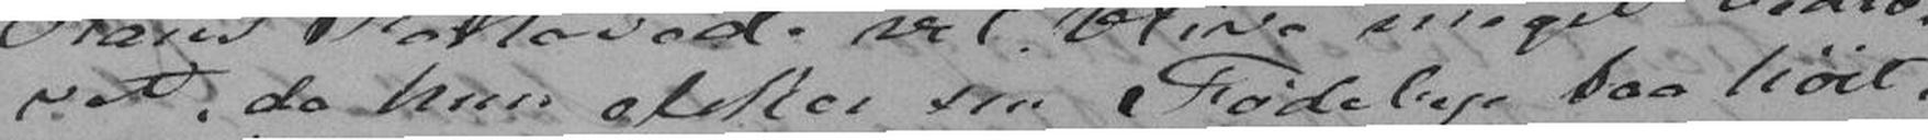vet, da hun elsker sin Fødeby saa høit
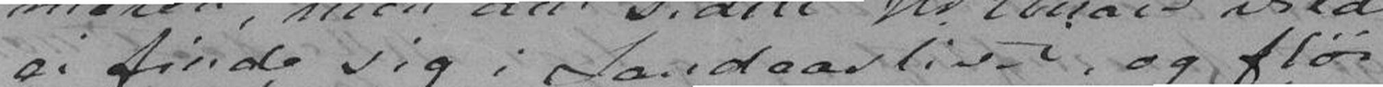ei finde sig i Landaaslivet, og fløi
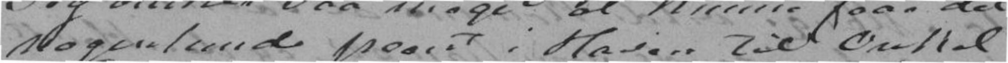nogenlunde peent i Haven til Onkel
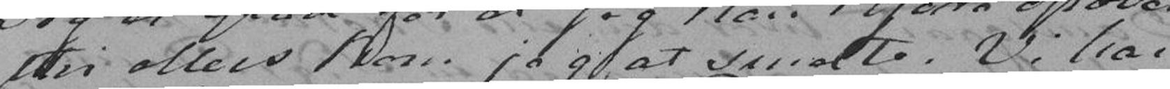thi ellers kom jeg at smelte. Vi har
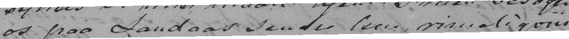os paa Landaas senere hen, rimeligviis
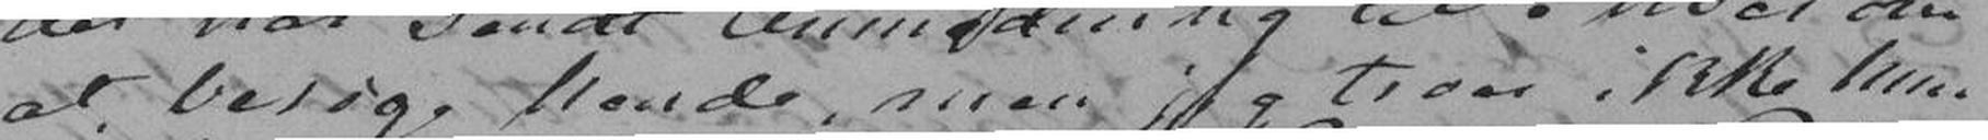at besøge hende, men jeg troer ikke hun
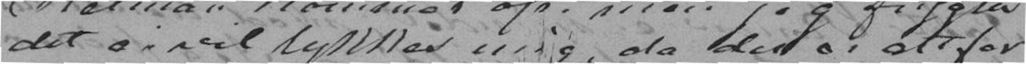det ei vil lykkes mig, da der er altfor
